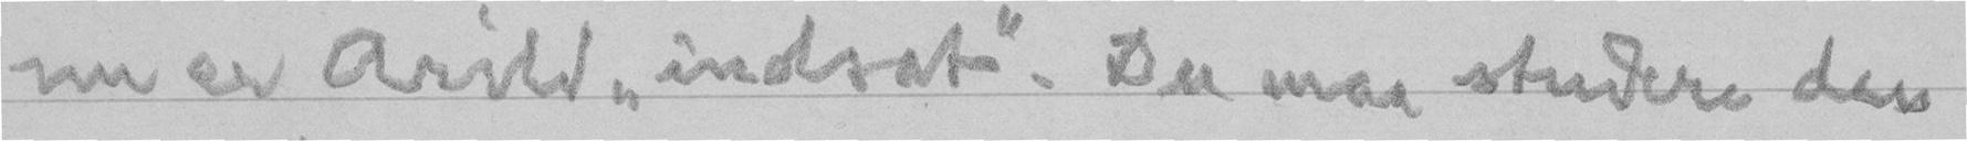nu er Arild "indsat". Du maa studere den
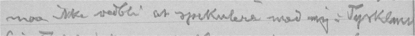maa ikke vedbli at spekulere mod mig i Tyskland
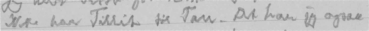ikke har Tillit til Tau. Det har jeg ogsaa
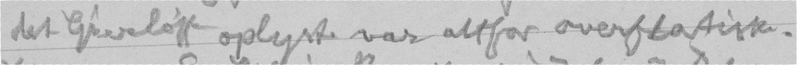det Gierløff oplyste var altfor overflatisk.
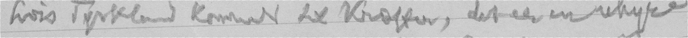hvis Tyskland kommer til Kræfter, det er en uhyre
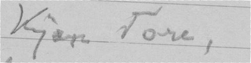Kjære Tore,
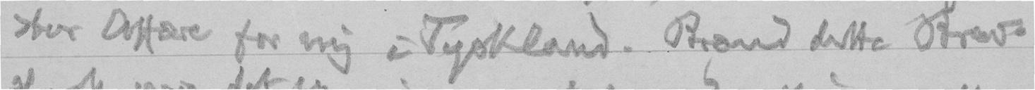stor Affære for mig i Tyskland. Brænd dette Brev.
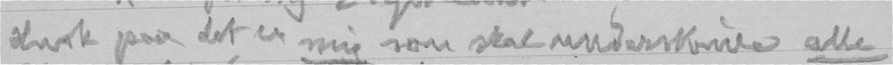Husk paa det er mig som skal underskrive alle
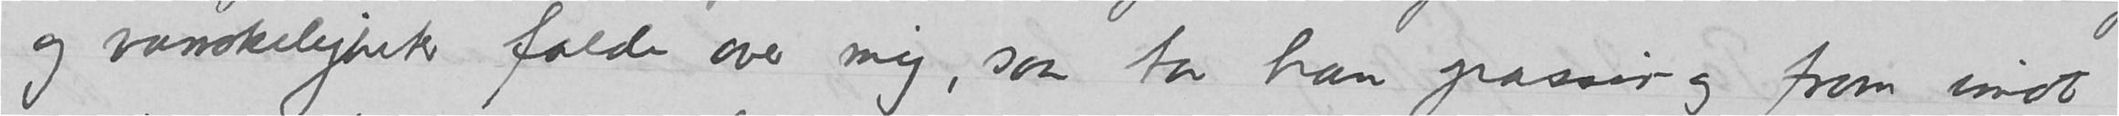og vanskeligenter falde over mig, saa ta han passer og from imot
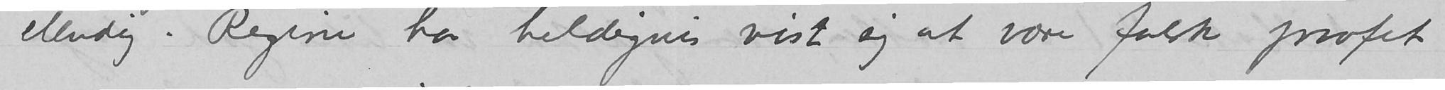elendig. Regine har heldigvis vist sig at være falsk profet
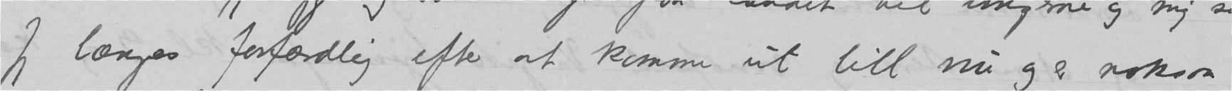Jeg længes forfærdelig efter at komme ut litt nu og er noksaa
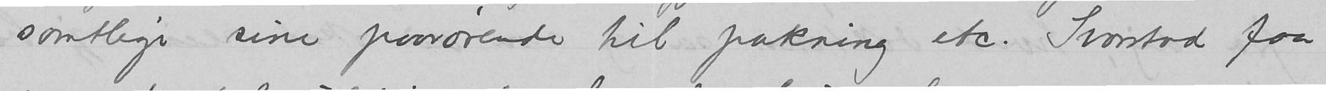samtlige sine paarørende til pakning etc. Svarstad faar
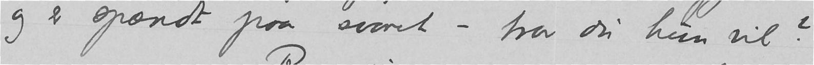og er spændt paa svaret – tror du hun vil?
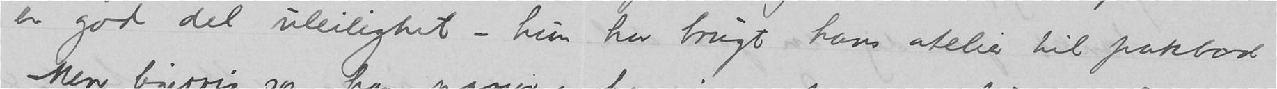en god del uleilighet – hun har brugt hans atelier til pakbod
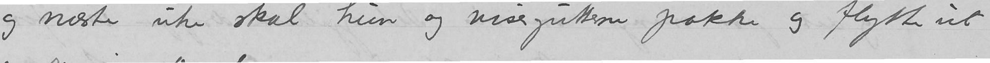og næste uke skal hun og visergutterne pakke og flytte ut
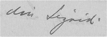din Sigrid
